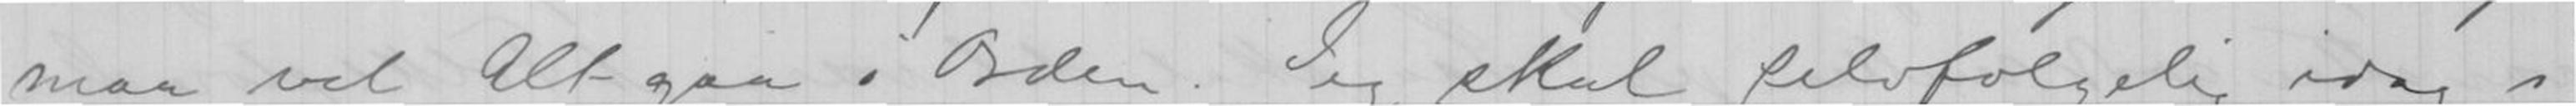maa vel Alt gaa i Orden. Jeg skal selvfølgelig idag i
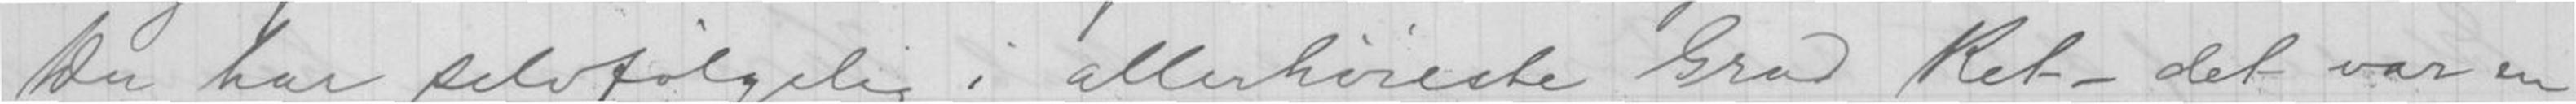Du har selvfølgelig i allerhøieste Grad Ret - det var en
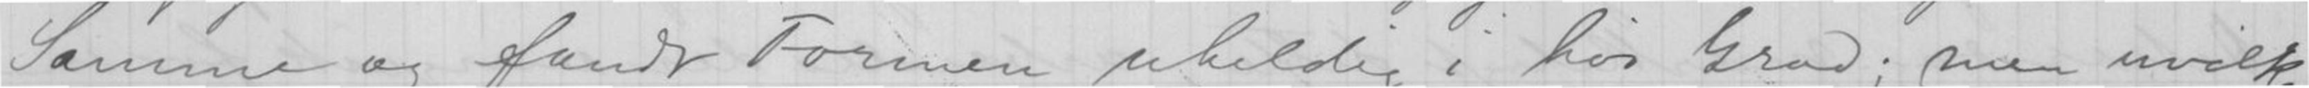Samme og fandt Formen uheldig i høi Grad; men uvilkaar-
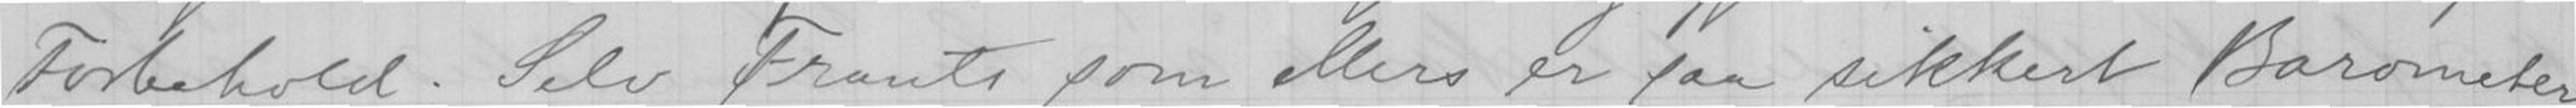Forbehold. Selv Frants som ellers er saa sikkert Barometer
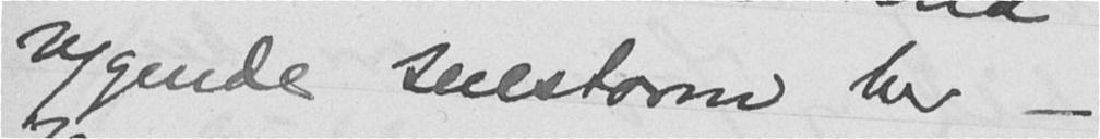rygende snestorm her -
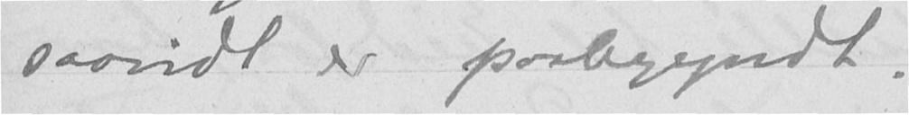saavidt er paabegyndt.
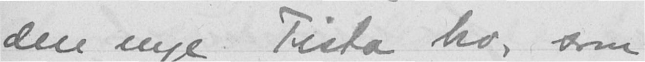den nye Tista bro, som
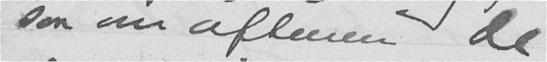saa om aftenen de
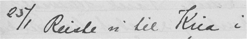25/1 Reiste vi til Kria i
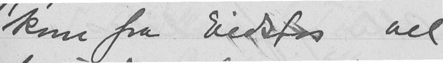kom fra Eidsfos vel
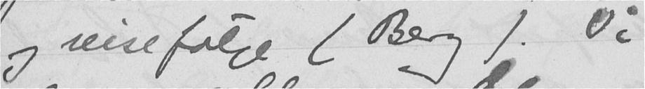og reisefølge (Berg). Vi
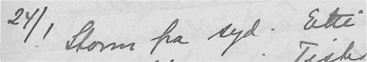24/1 Storm fra syd. Etti
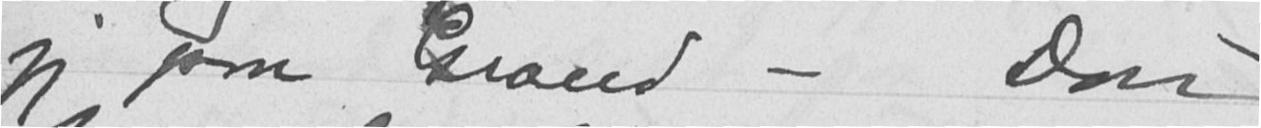jeg paa Grand - Don
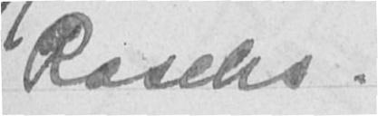Raschs.
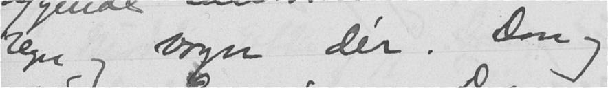regn og vogn dér. Don og
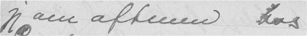jeg om aftenen hos
